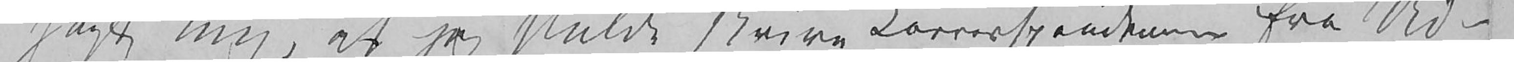sagt mig, at jeg skulde skrive Korresspondencer fra Ud=
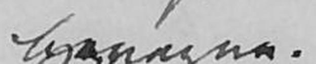beregne
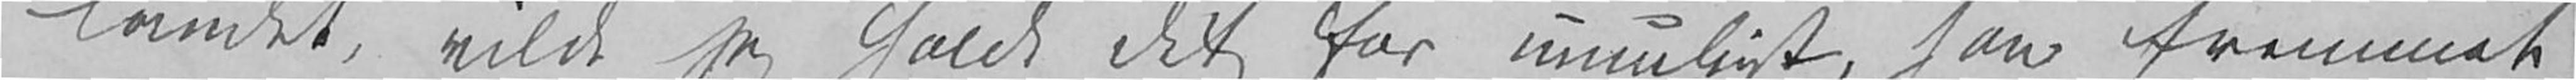landet, vilde jeg holdt det for umuligt, saa fremmet
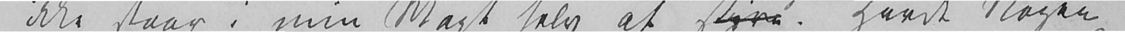ikke staar i min Magt selv at styre. Havde Nogen
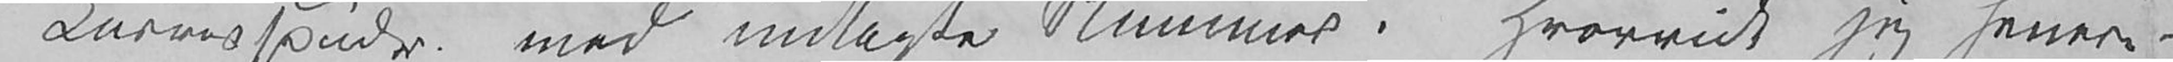Korresspndr. med indlagte Nummer. Hvorvidt jeg senere
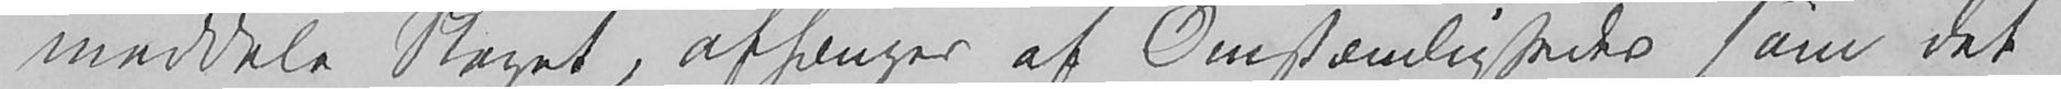meddele Noget, afhænger af Omstændigheder som det
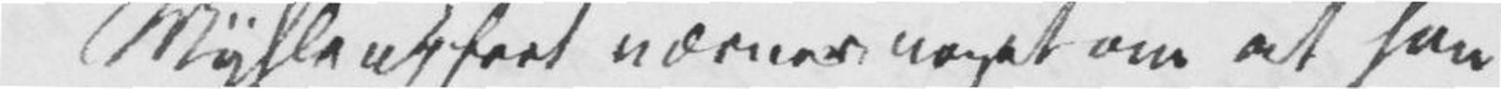Myhlenphort nævner noget om at han
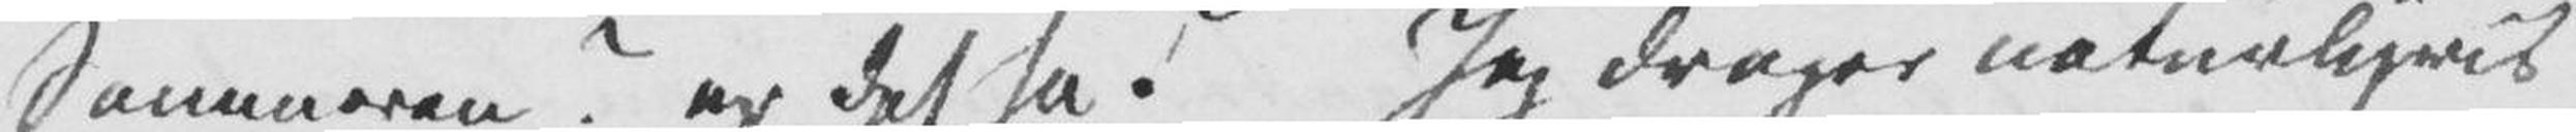Sommeren»? er det så? Jeg drager naturligvis
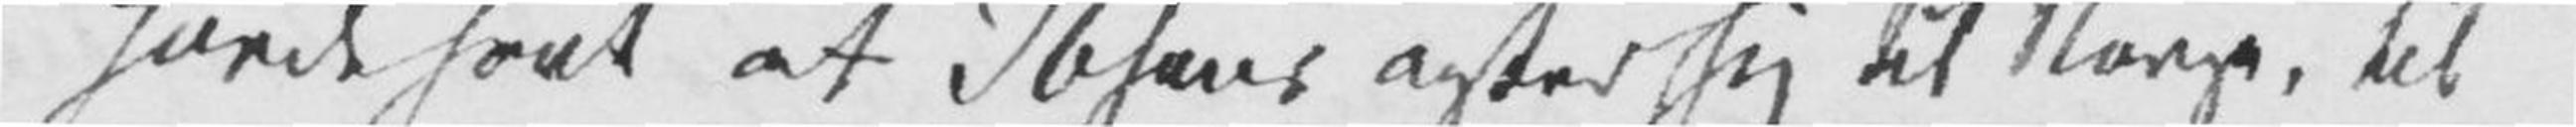havde hørt at «Ibsens agter sig til Norge, til
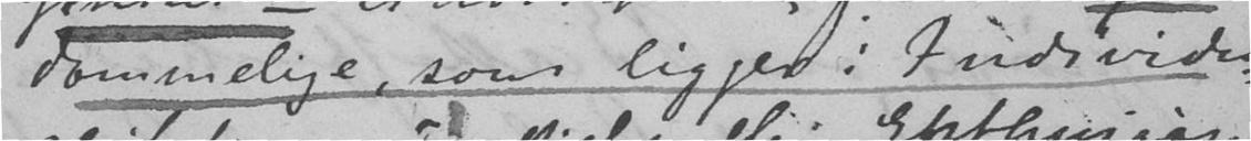dommelige, som ligger i Individu-
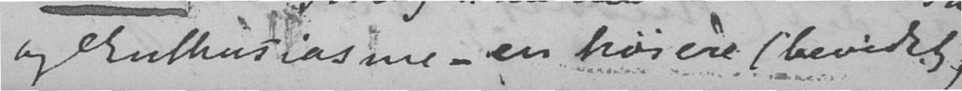og luthusiasme - en høiere (bevidst)
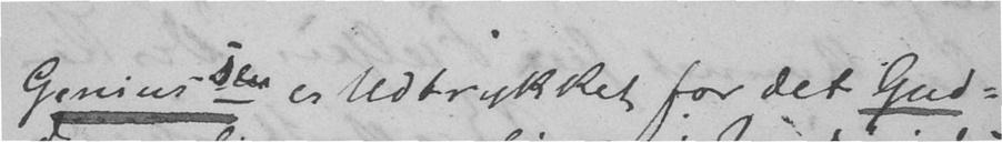Genius er Udtrykket for det Gud-
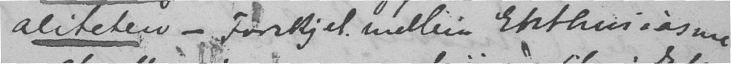aliteten - Forskjel mellem Enthusiasme
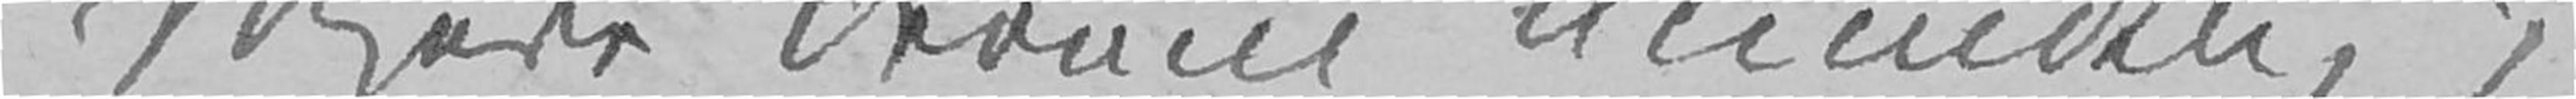Mere derom mundtlig;
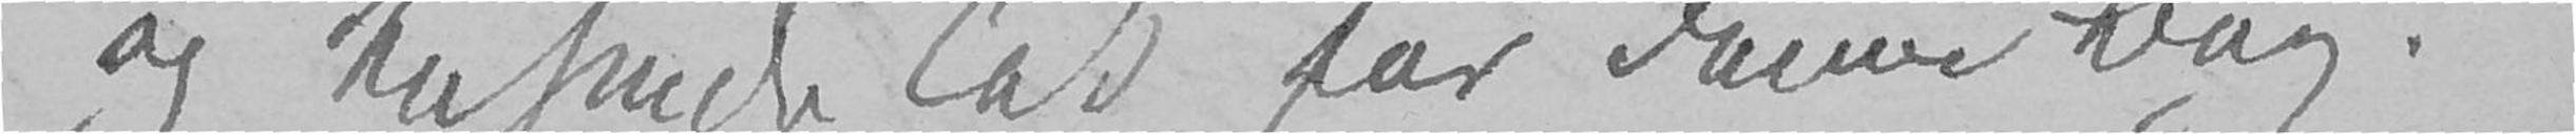og tusinde Tak for denne Bog.
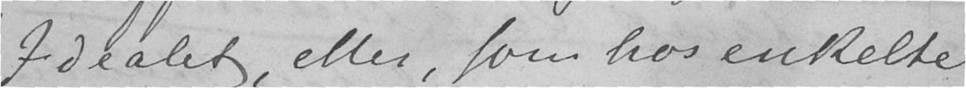Idealet, eller, som hos enkelte
